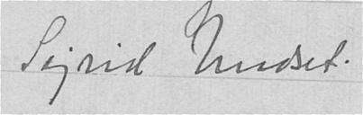Sigrid Undset.
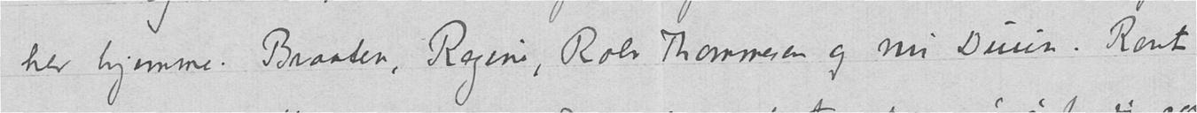her hjemme.  Braaten, Regine, Rolv Thommesen og nu Duun. Rent
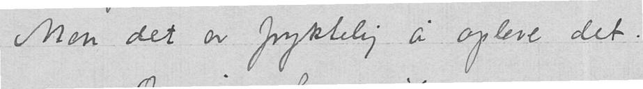Men det er fryktelig å opleve det.
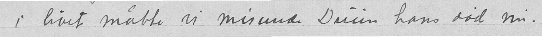i livet måtte vi misunde Duun hans død nu.
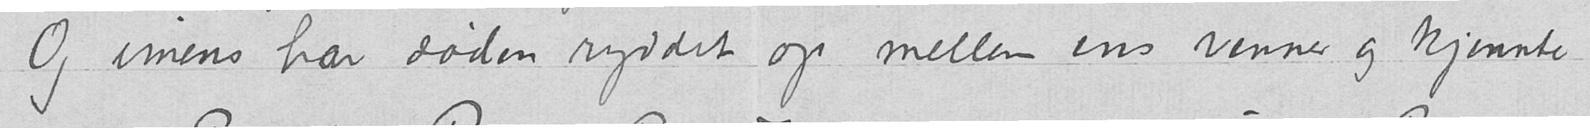Og imens har døden ryddet op mellem ens venner og kjennte
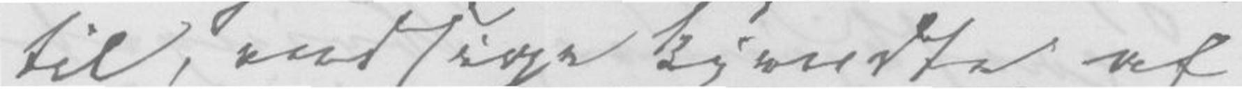til, endsige kjendte af
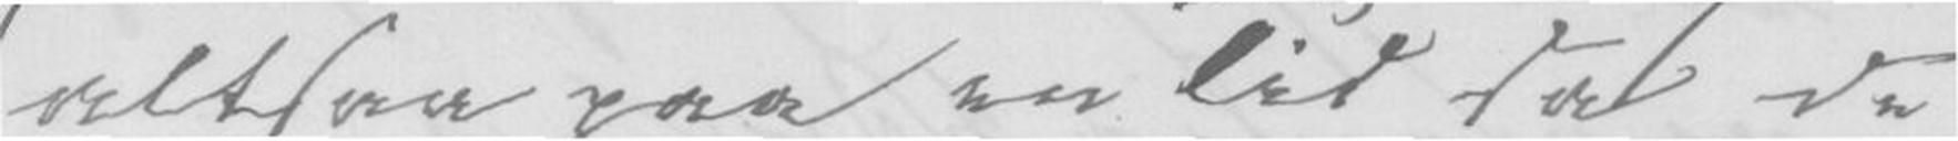altsaa gaa en Tid da de
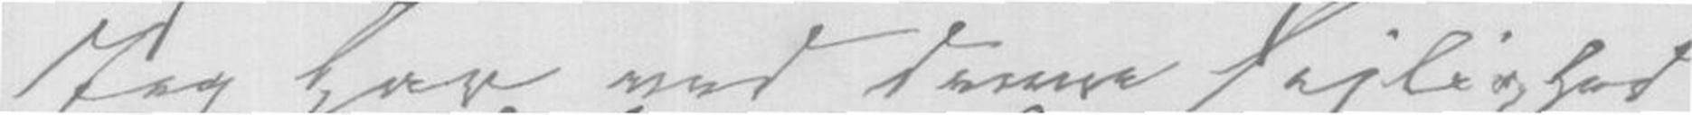Jeg har ved denne Lejlighed
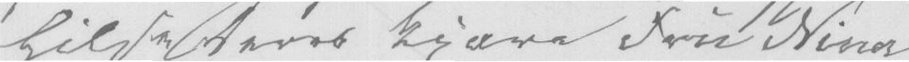hilse Deres kjære Fru Nina
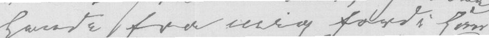hende fra mig fordi Han
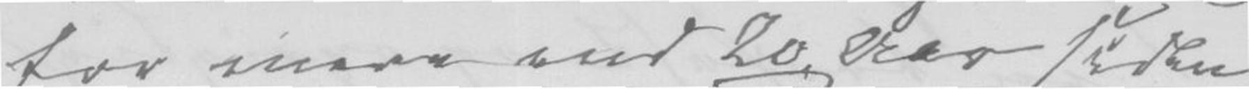for mere end 20 Aar siden,
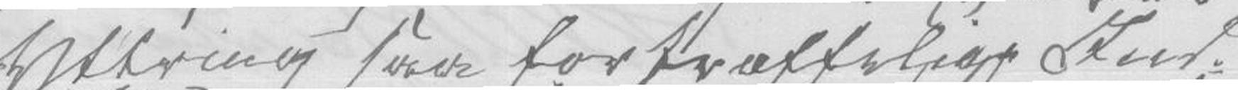Yttring saa fortræffelige Ind-
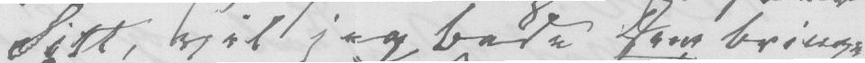Sitt, vil jeg bede Dem bringe
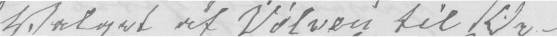Valget af Vølven til Op-
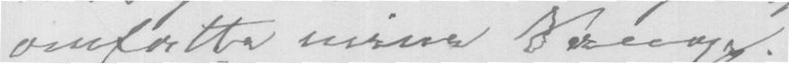omfatte mine Sange.
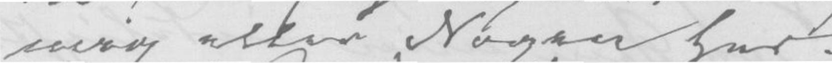mig etter Nogen her.
